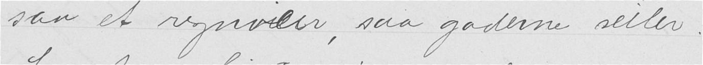saa et regnveir, saa gaderne seiler.
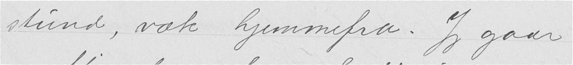stund, væk hjemmefra. Jeg gaar
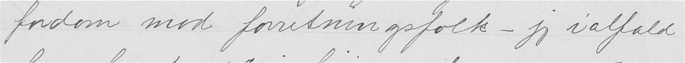fordom mod forretningsfolk - jeg ialfald
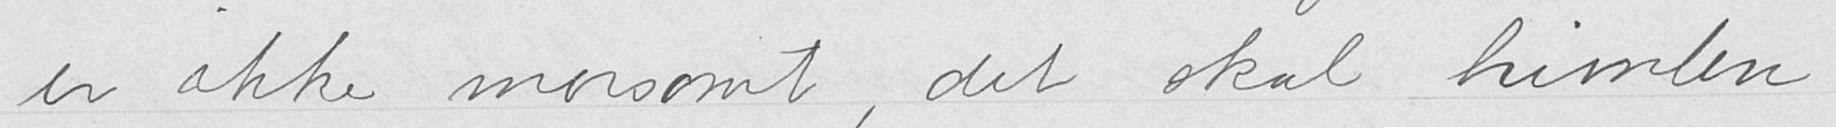er ikke morsomt, det skal himlen
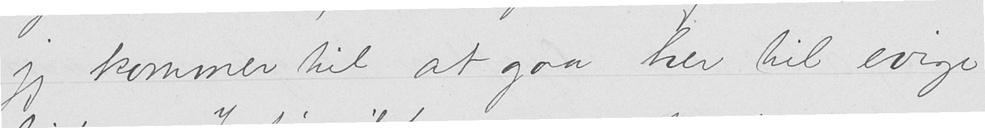jeg kommer til at gaa her til evige
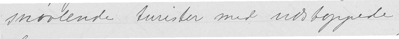snøvlende turister med udstoppede
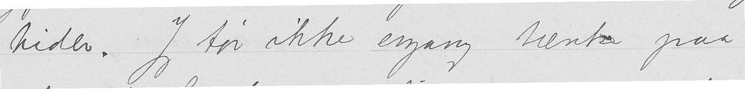tider. Jeg tør ikke engang tænke paa
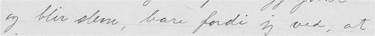og blir slem, bare fordi jeg ved, at
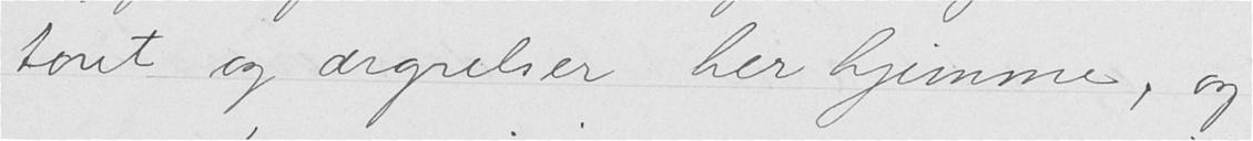toret og ærgrelser her hjemme, og
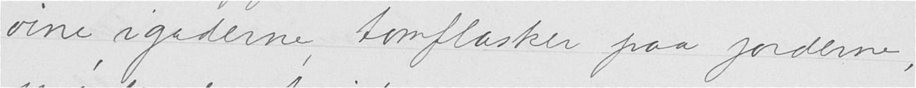øine i gaderne, tomflasker paa jorderne,
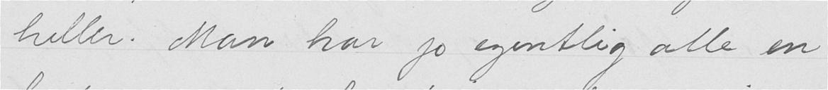heller. Man har jo egentlig alle en
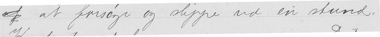at forsøge og slippe ud en stund.
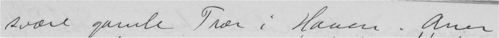svære gamle Trær i Haven. Aner
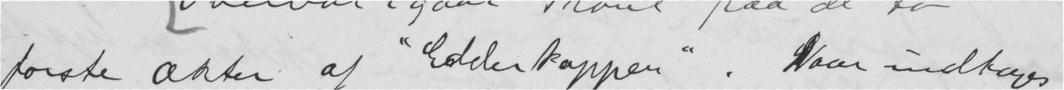Første Akter af "Edderkoppen". Naar indtages
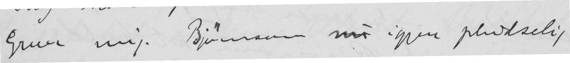Gruer mig. Bjørnson nu igjen pludselig
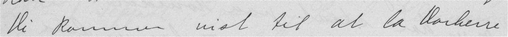Vi kommer vist til at la Vorherre
